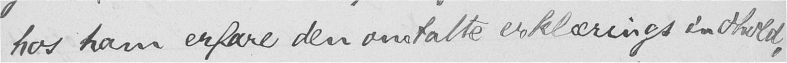hos ham erfare den omtalte erklærings indhold,
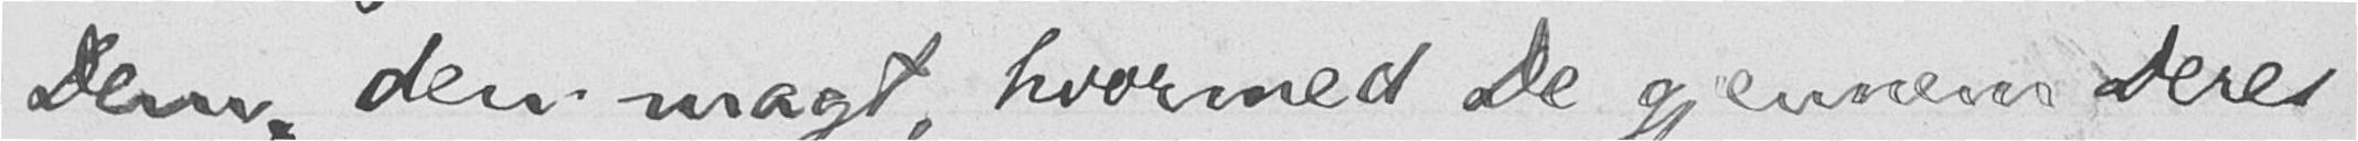Dem, den magt, hvormed De gjennem Deres
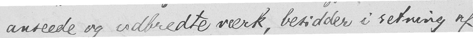anseede og udbredte værk, besidder i retning af
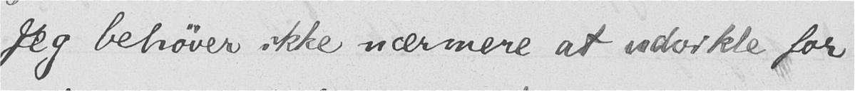Jeg behøver ikke nærmere at udvikle for
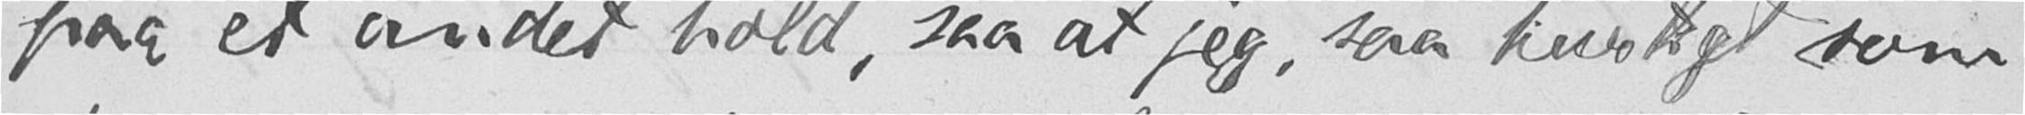paa et andet hold, saa at jeg, saa hurtigt som
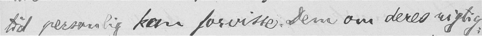tid personlig kan forvisse Dem om deres rigtig-
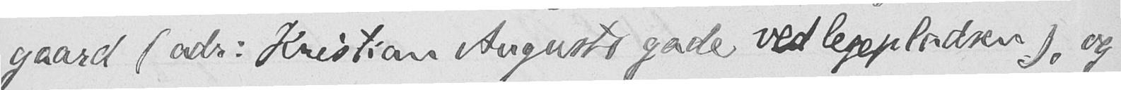gaard (adr: Kristian Augusts gade ved legepladsen), og
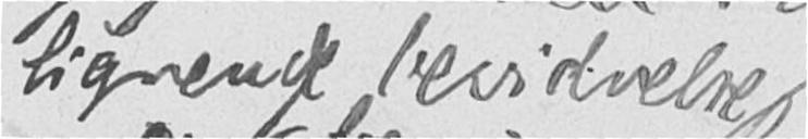lignende bevidnelse
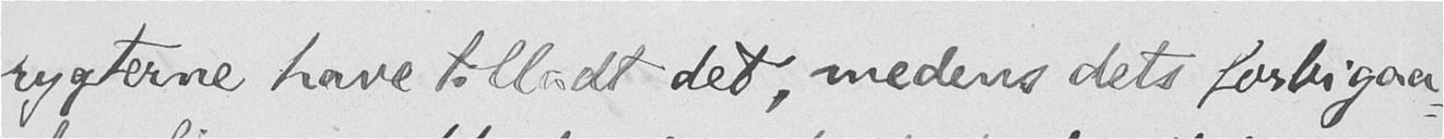rygterne have tilladt det, medens dets forbigaa-
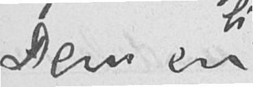Dem en
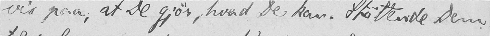vis paa, at De gjør, hvad De kan. Støttende Dem
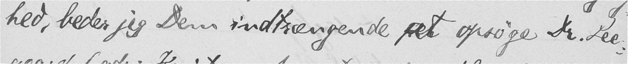hed, beder jeg Dem indtrængende ret opsøge Dr. Lee-
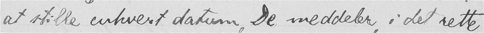at stille enhvert datum, De meddeler, i det rette
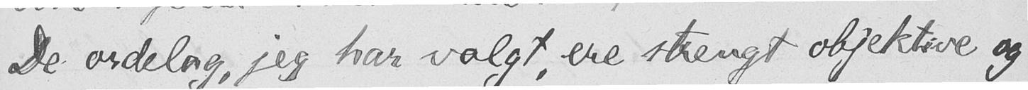De ordelag, jeg har valgt, ere strengt objektive og
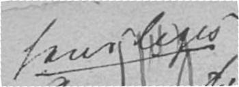san ages
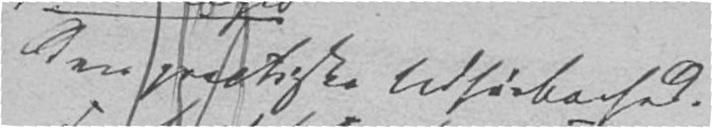Den practiske Udførbarhed.
